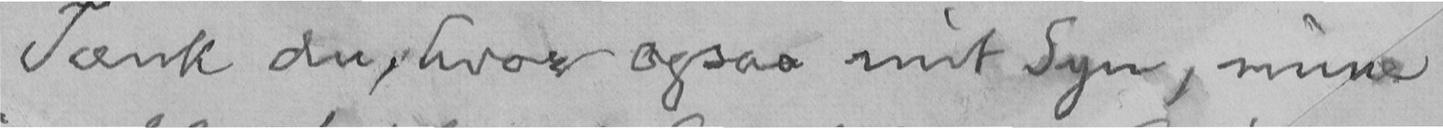Tænk du, hvor ogsaa mit Syn, mine
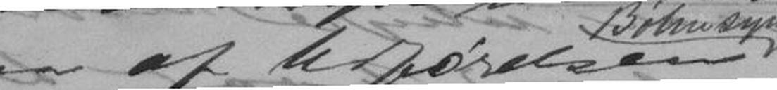faa af Udførelsen
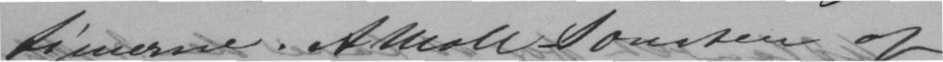timerne. AMoll Sonaten af
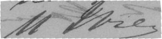H Ibsen
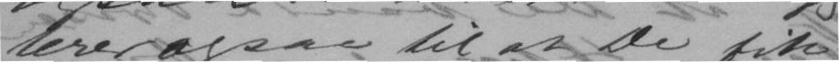lerer ogsaa til at De fik
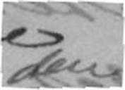den
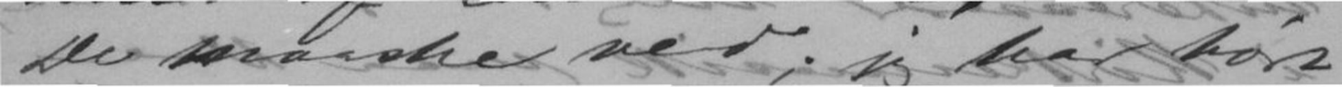De maaske ved; jeg har hørt
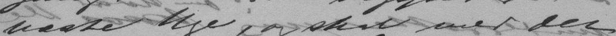næste Uge, og skal med det
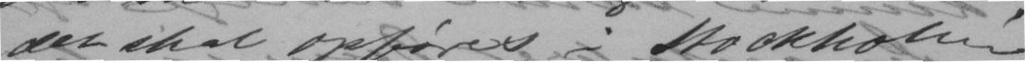det skal opføres i Stockholm
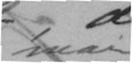man
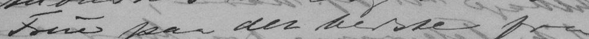Frue paa det bedste fra
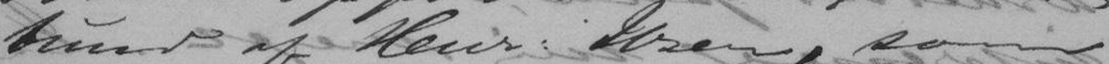bund" af Henr. Ibsen, som
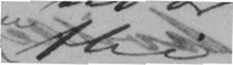thi
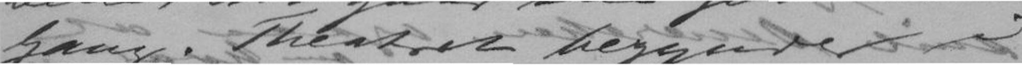Gang. Theatret begynder i
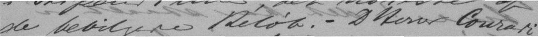de bevilgede Beløb. - D Herrer Conradi
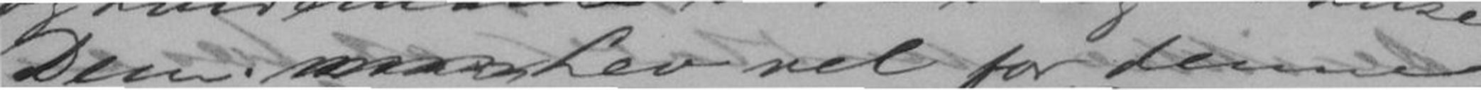Dem. Lev vel for denne
